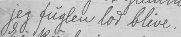jeg fuglen lod blive.
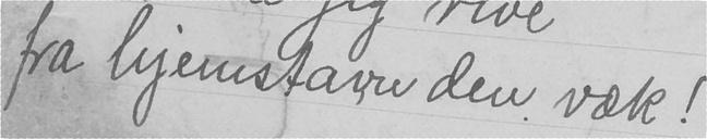fra hjemstavn den væk!
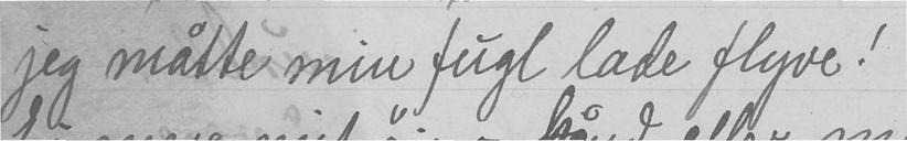jeg måtte min fugl lade flyve!
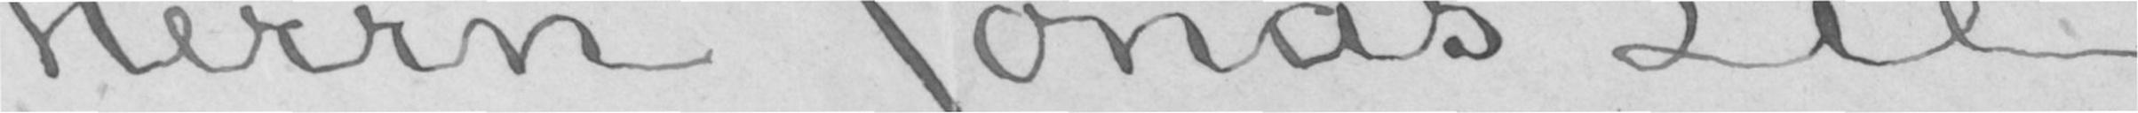Herrn Jonas Lie
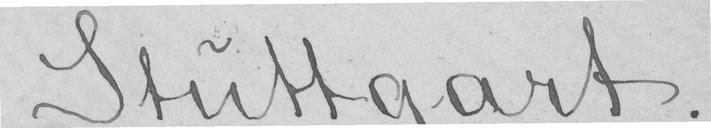Stuttgart.
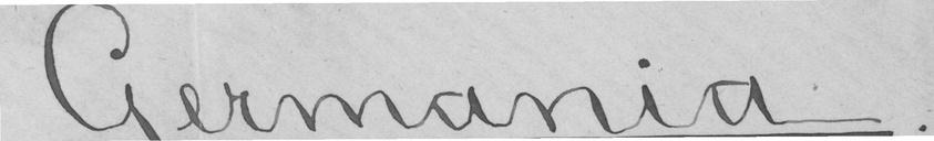Germania.
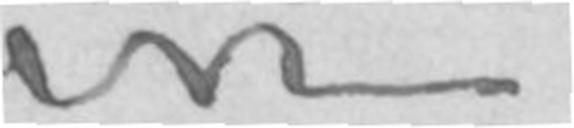in
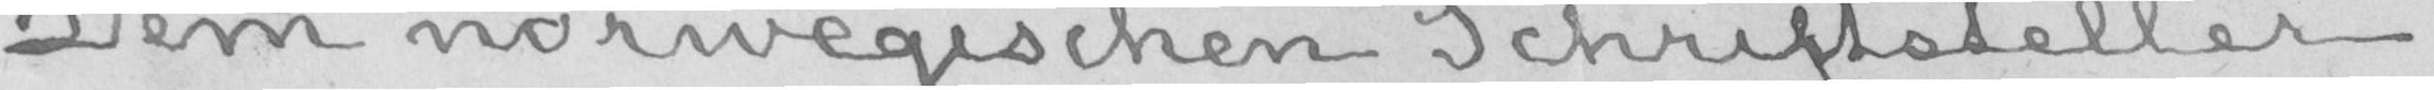Dem norwegischen Schriftsteller
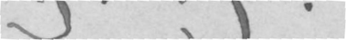z. Z.
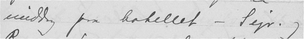middag paa hotellet - Sejr. og
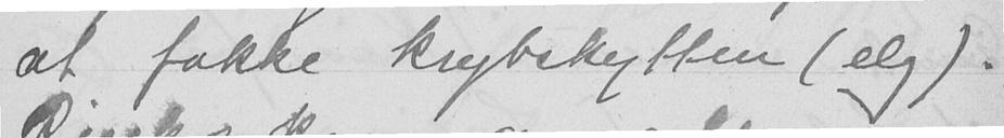at fakke krybskytten (elg).
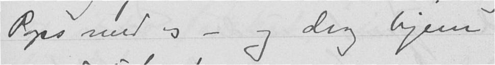Rops med os - og drog hjem
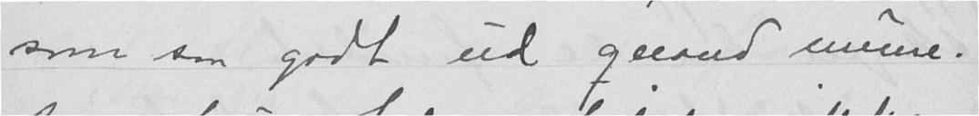som saa godt ud quand même.
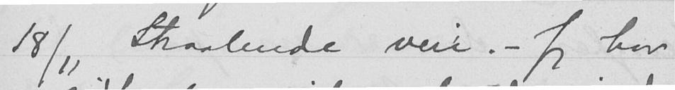18/11 Straalende veir. - Jeg har
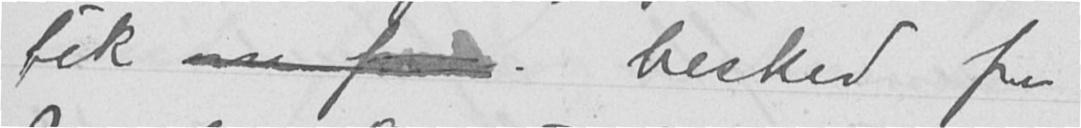fik om fer. besked fra
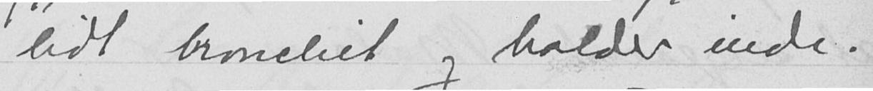lidt bronchit og holder inde.
